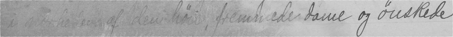i nærheden af den høie, fremmede dame og ønskede
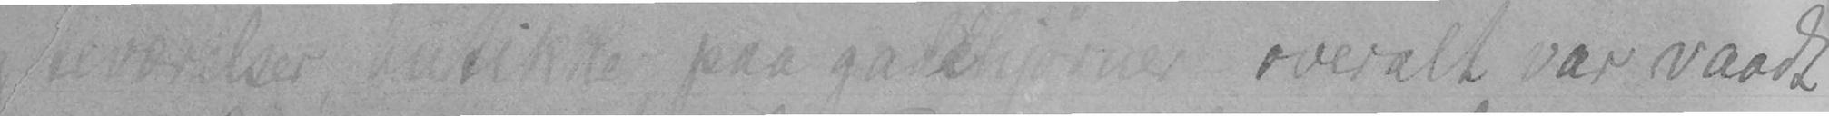leværelser, kutikke, paa gadehjørner overalt var vaadt
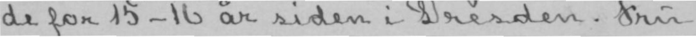de for 15-16 år siden i Dresden. Fru
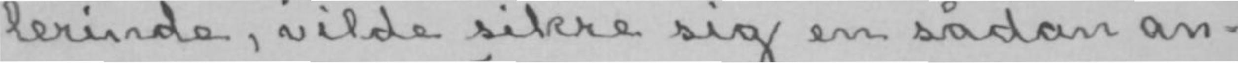lerinde, vilde sikre sig en sådan an-
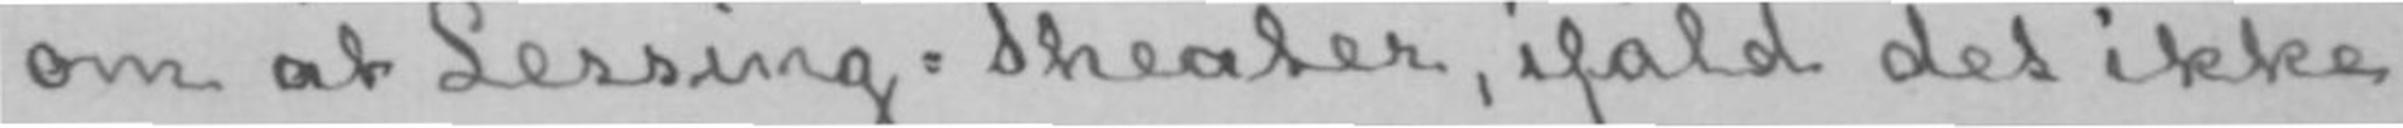om at Lessing-Theater, ifald det ikke
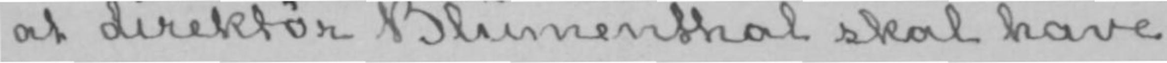at direktør Blumenthal skal have
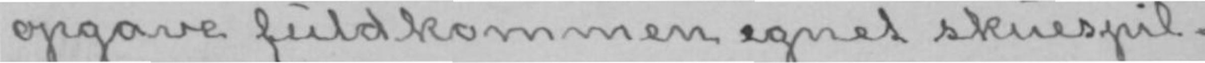opgave fuldkommen egnet skuespil-
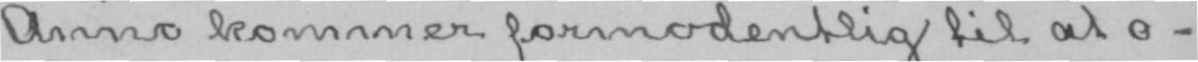Anno kommer formodentlig til at o-
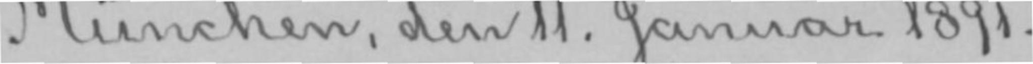München, den 11. Januar 1891.
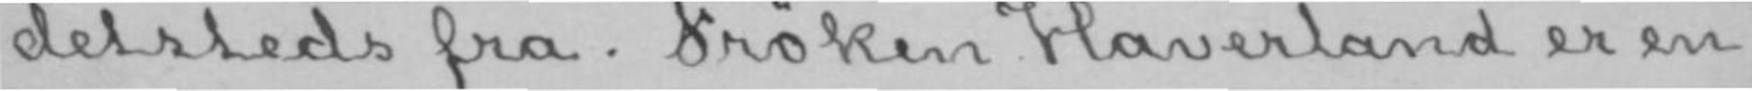detsteds fra. Frøken Haverland er en
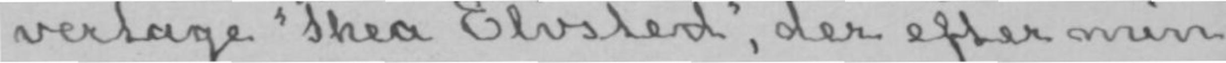vertage «Thea Elvsted», der efter min
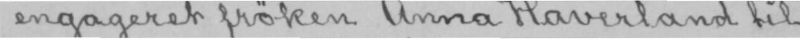engageret frøken Anna Haverland til
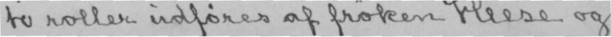to roller udføres af frøken Heese og
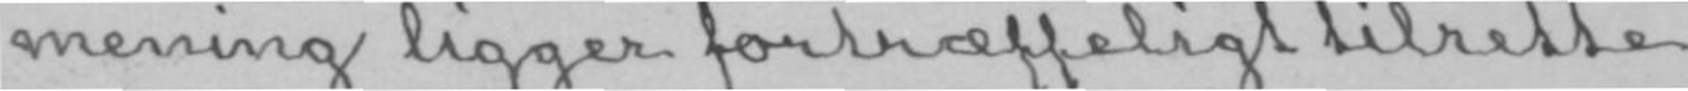mening ligger fortræffeligt tilrette
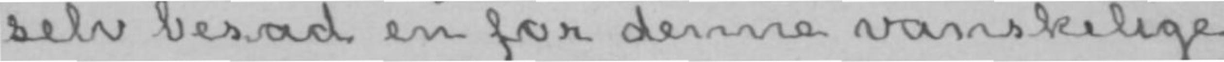selv besad en for denne vanskelige
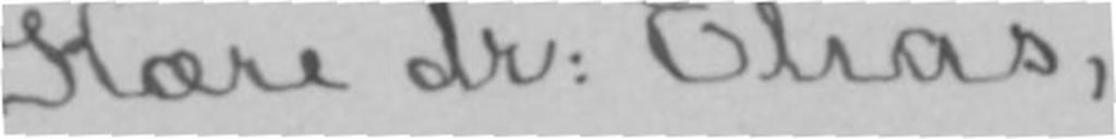Kære dr: Elias,
